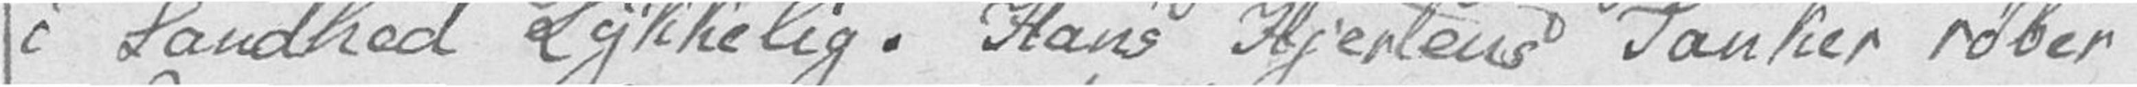i Sandhed Lykkelig. Hans Hjertens Tanker røber
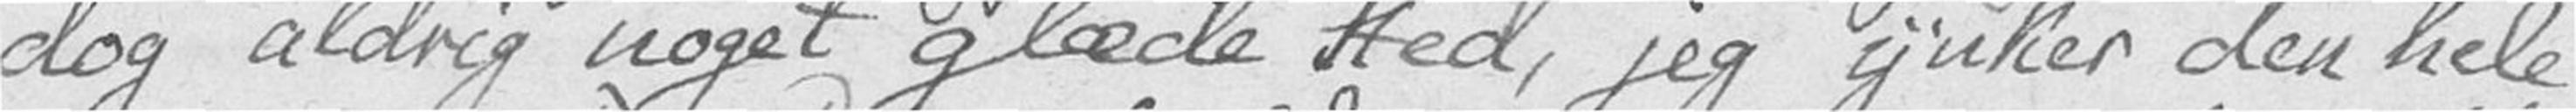dog aldrig noget glæde Sted, jeg ynker den hele
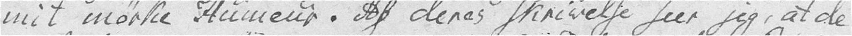mit mørke Humeur. Af deres skrivelse seer jeg, at de
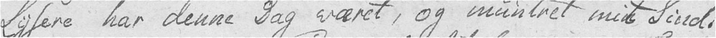Lysere har denne Dag været, og muntret mit Sind.
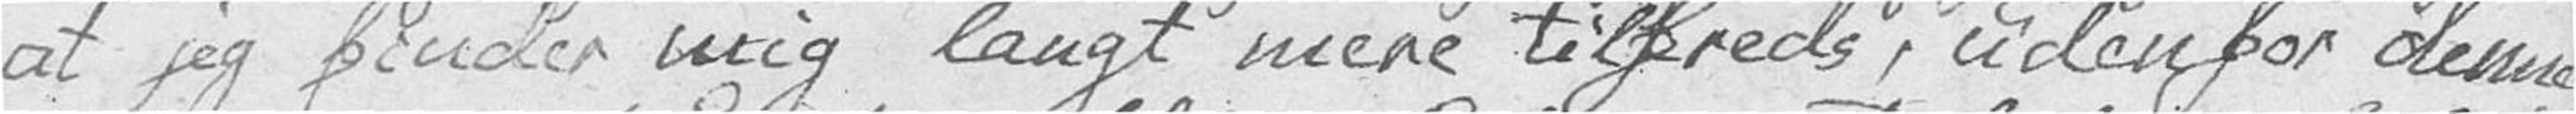at jeg finder mig langt mere tilfreds, udenfor denne
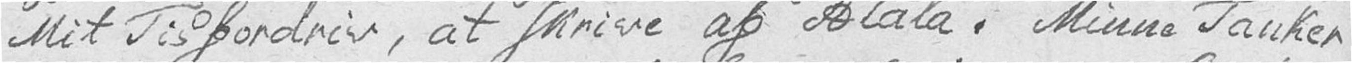Mit Tisfordriv, at skrive af Atala. Minne Tanker
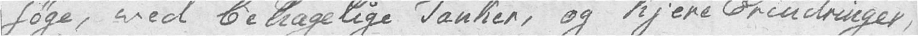søge, ved behagelige Tanker, og kjere Erindringer,
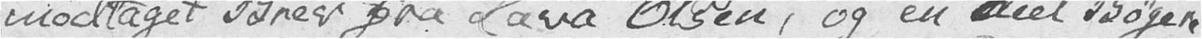modtaget Brev fra Lava Olsen, og en deel Bøger.
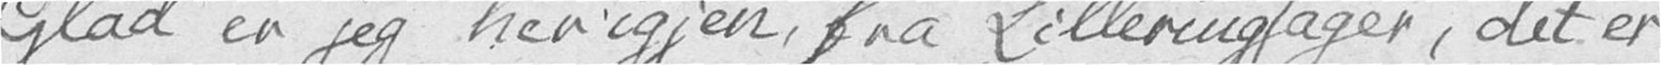Glad er jeg her igjen, fra Lilleringsager, det er
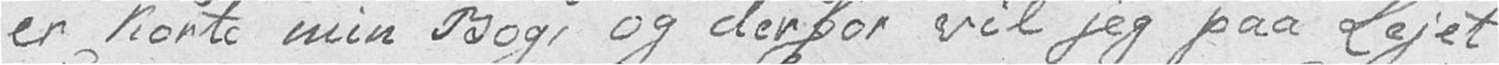er korte min Bog, og derfor vil jeg paa Lejet
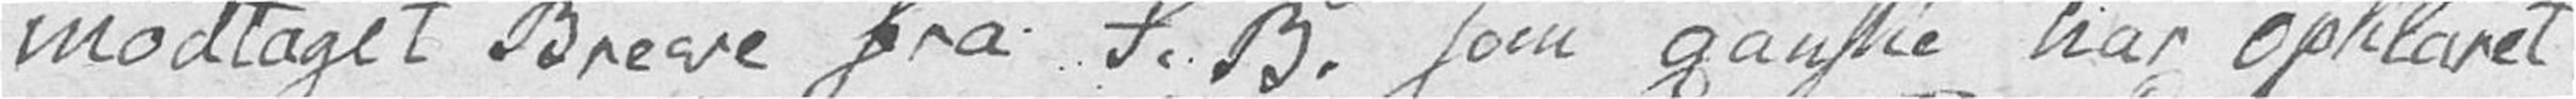modtaget Breve fra S. B. som ganske har opklaret
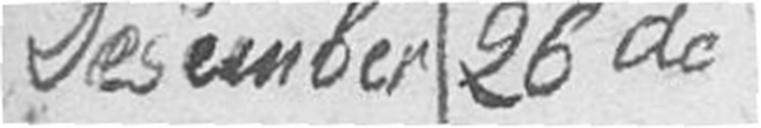Desember 26de
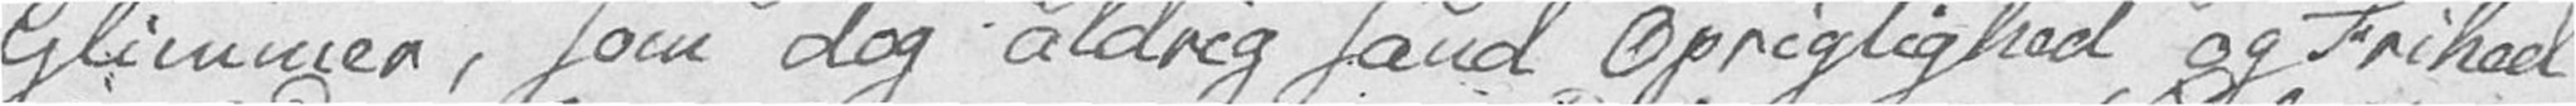Glimmer, som dog aldrig sand Oprigtighed og Frihed
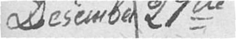Desember 27de
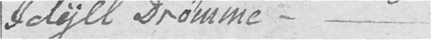Idyll Drømme. –
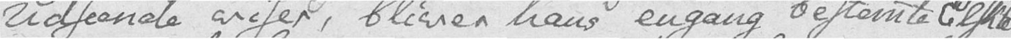Udseende viser, bliver hans engang bestemte Elskte
